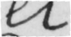er
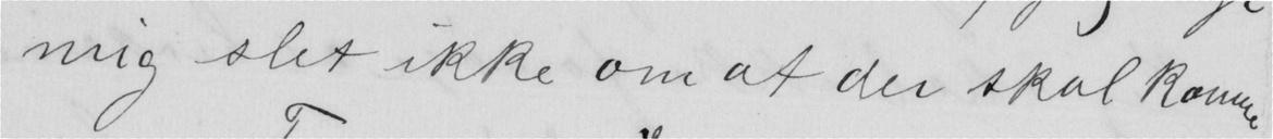mig slet ikke om at der skal komme
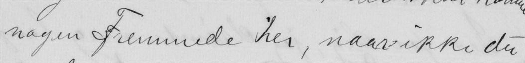nogen Fremmede der naar ikke du
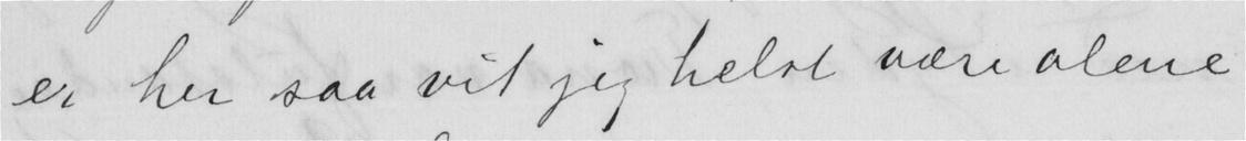er her saa vil jeg helst være alene
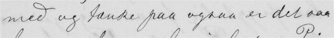med og tænke paa ogsaa er det saa
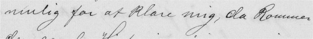nemlig for at klare mig, da Kommer
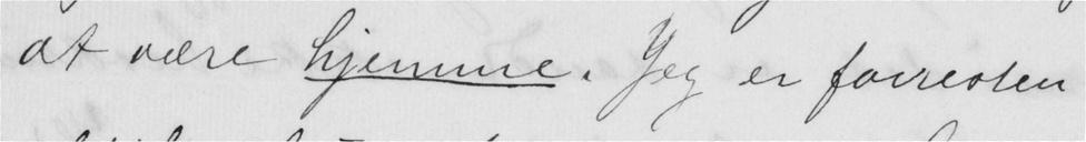at være hjemme. Jeg er forresten
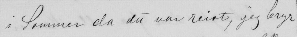i Sommer da du var reist, jeg bryr
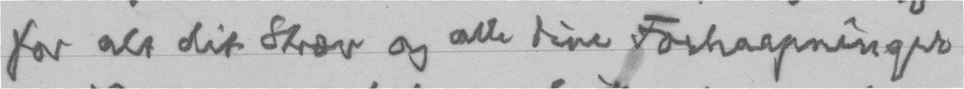for alt dit Stræv og alle dine Forhaapninger
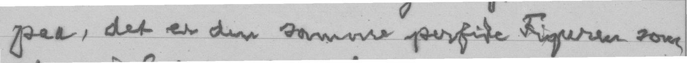paa, det er den samme perfide Figuren som
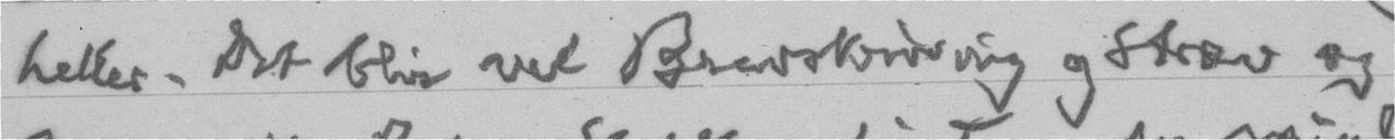heller. Det blir vel Brevskrivning og Stræv og
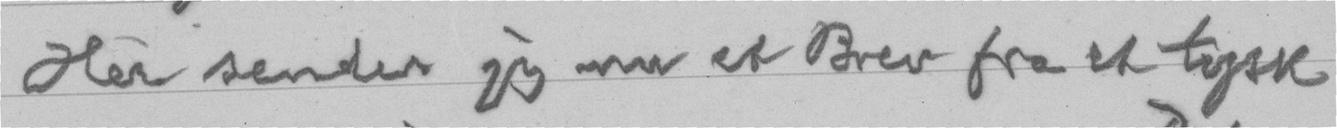Har sender jeg nu et Brev fra et tysk
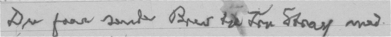Du faar sende Brev til Fru Stray med
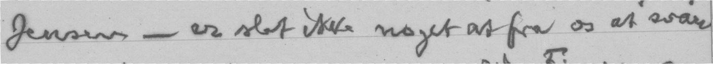Jensen - er slet ikke noget at fra os at svare
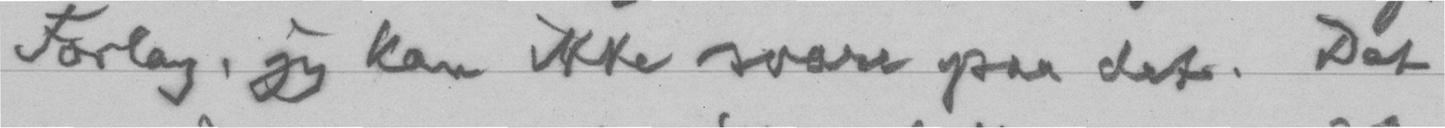Forlag, jeg kan ikke svare paa det. Det
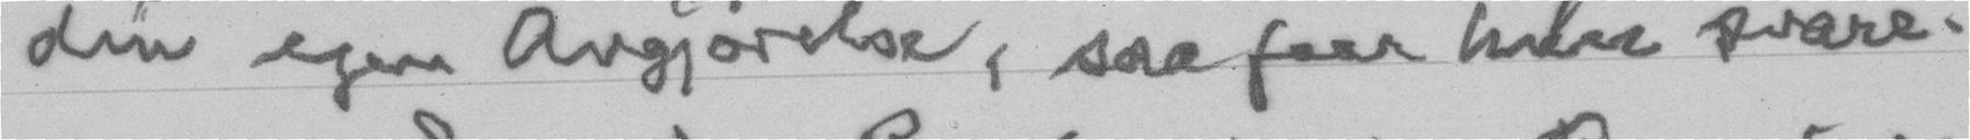din egen Avgjørelse, saa faar hun svare.
